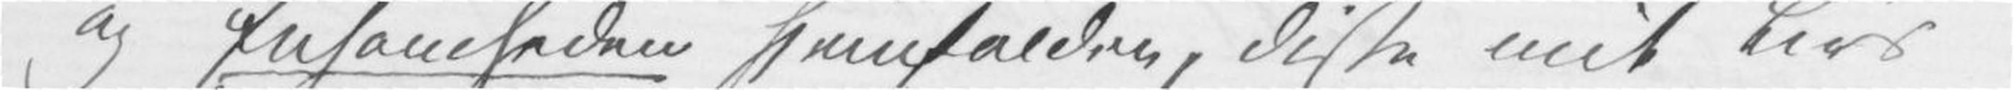og Ensomheden hjemfalden, disse mit Livs
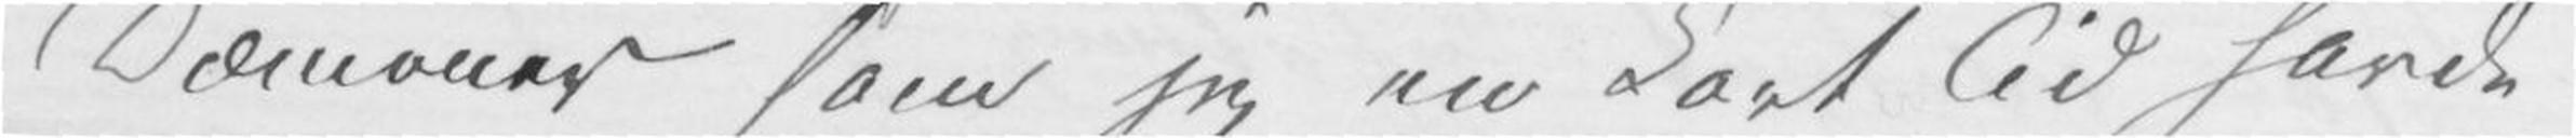Dæmoner som jeg en kort Tid havde
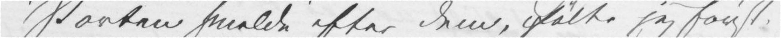«Porten smelde» efter Dem, følte jeg først
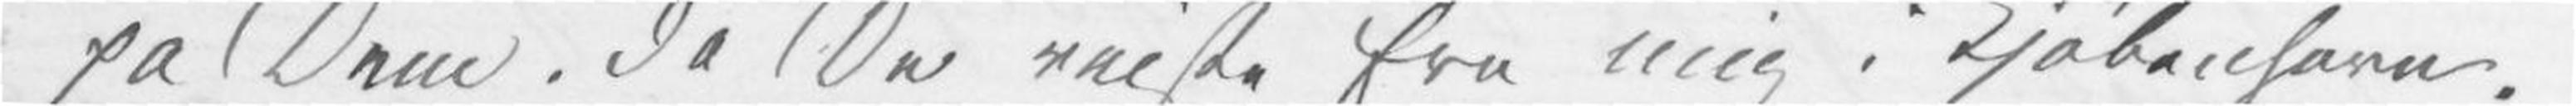på Dem, da De reiste fra mig i Kjøbenhavn.
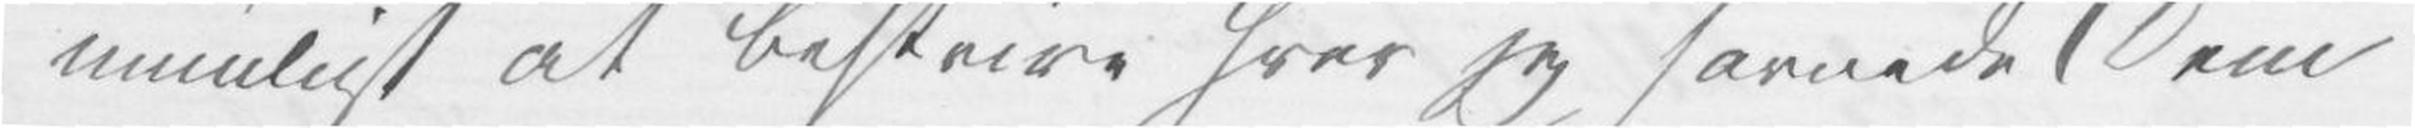umuligt at beskrive hvor jeg savnede Dem
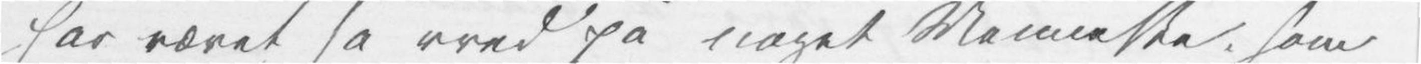har været så vred på noget Menneske, som
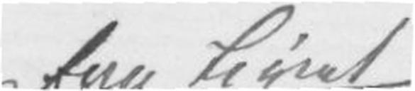fra Livet
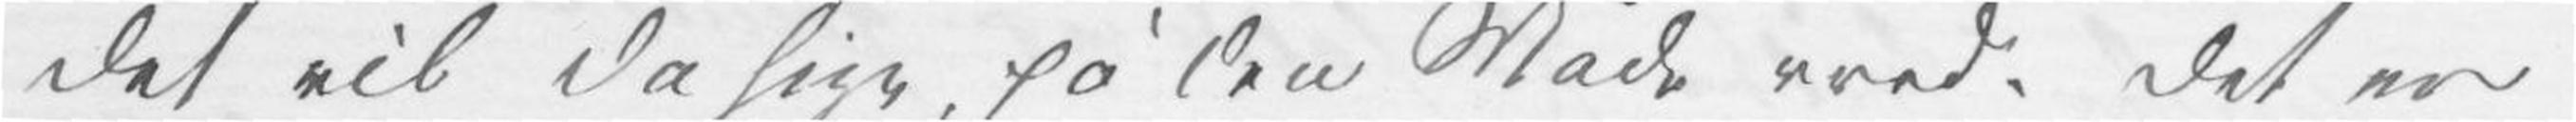Det vil da sige, på den Måde vred. Det er
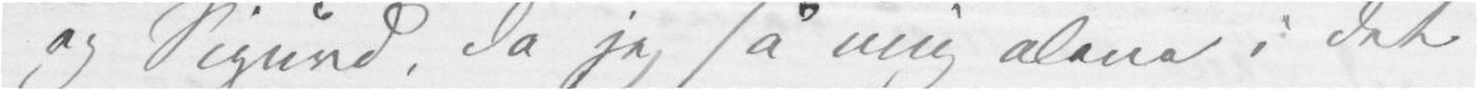og Sigurd, da jeg så mig alene i det
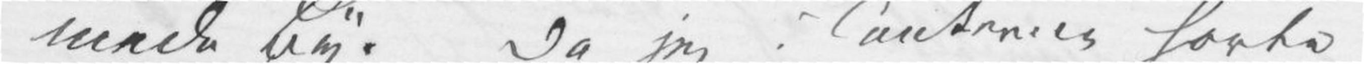mede By. Da jeg i Tankerne hørte
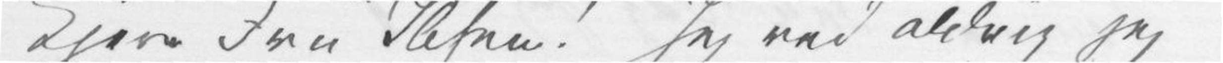Kjere Fru Ibsen! Jeg ved aldrig jeg
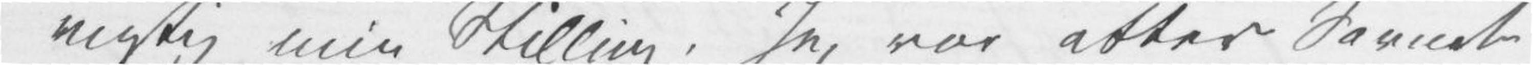rigtig min Stilling. Jeg var atter Savnet
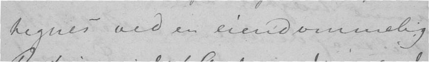tegnes ved en eiendommelig
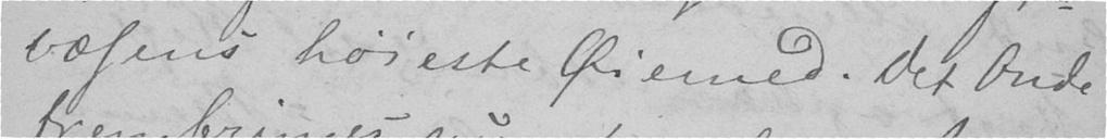væsens høieste Øiemed. Det Onde
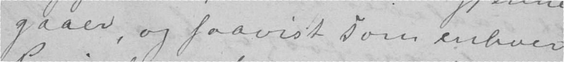gaaer, og saavist som enhver
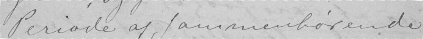Periode af sammenhørende
